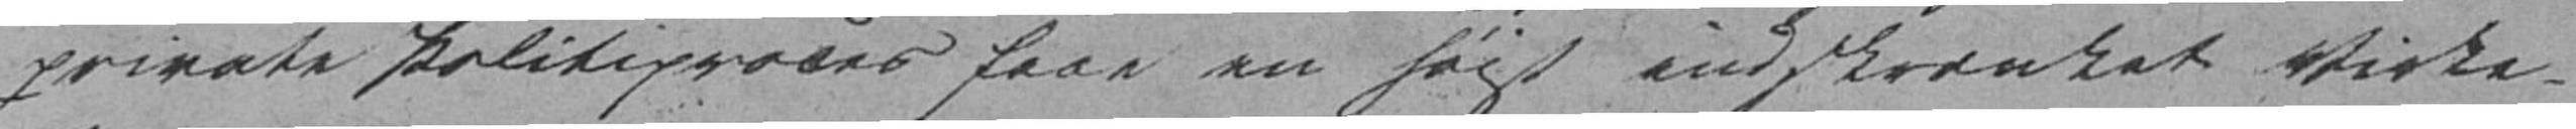private Politiproces faae en høist indskrænket Virke-
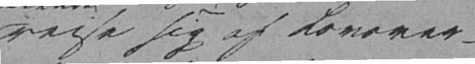reise sig af Lovover-
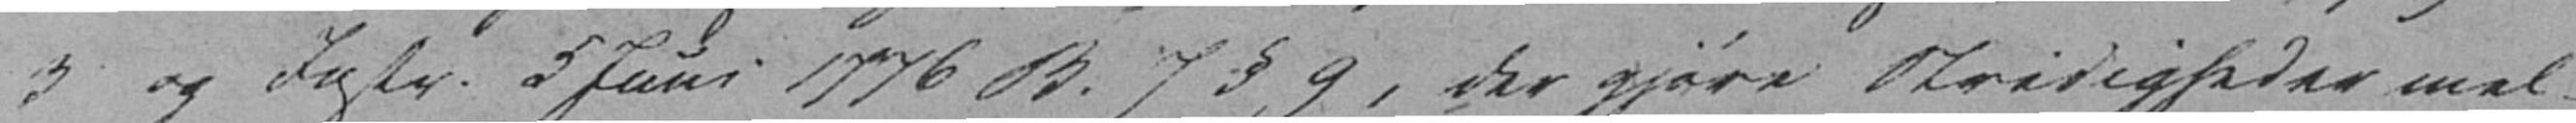3 og Instr. 5 Juni 1776 B. 7 § 9, der gjøre Stridigheder mel-
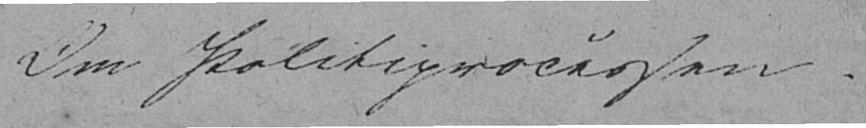Om Politiprocessen.
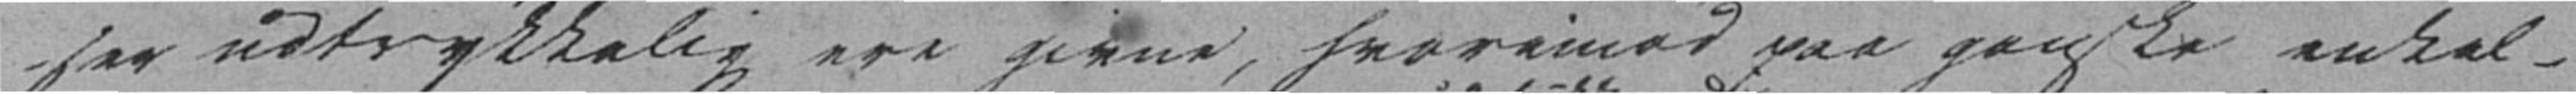ser udtrykkelig ere givne, hvorimod paa ganske enkel-
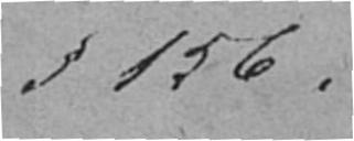§ 156.
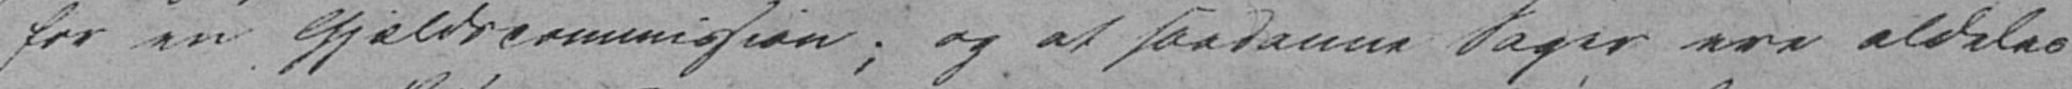for en Gjældscommission; og at saadanne Sager ere aldeles
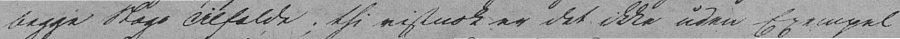begge Slags Tilfælde; thi vistnok er det ikke uden Exempel
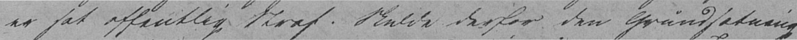er sat offentlig Straf. Skulde derfor den Grundsætning
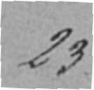23
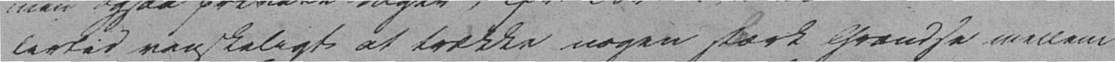lertid vanskeligt at trække nogen stærk Grændse mellem
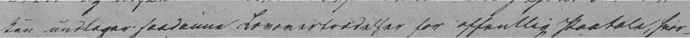kun undtager saadanne Lovovertrædelser for offentlig Paatale, hvor-
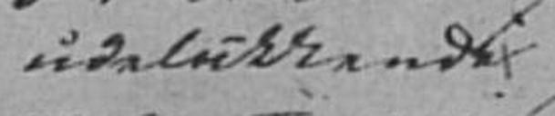udelukkende
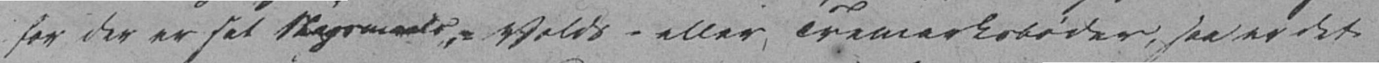for der er sat Slagsmaals, Volds- eller Tremarksbøder, saa er det
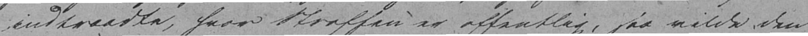indtraadte, hvor Straffen er offentlig, saa vilde den
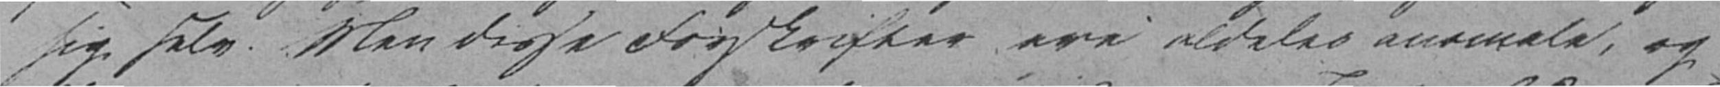sig selv. Men disse Forskrifter ere aldeles anomale, og
